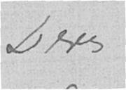Deres
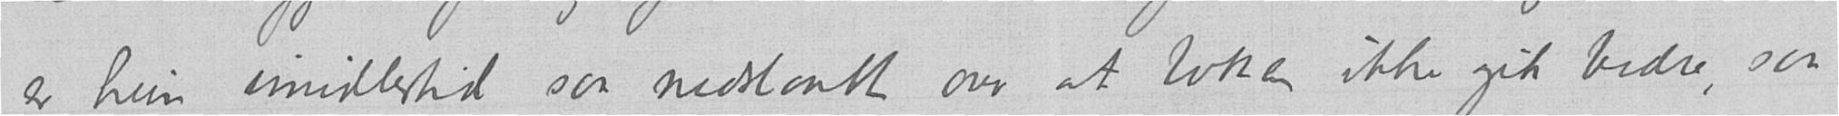er hun imidlertid saa nedslaatt over at boken ikke gik bedre, saa
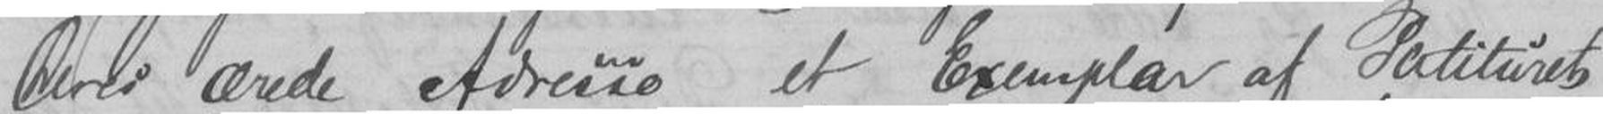Deres ærede Adresse et Exemplar af Patituret
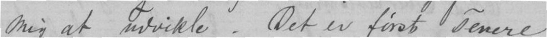mig at udvikle. Det er først senere
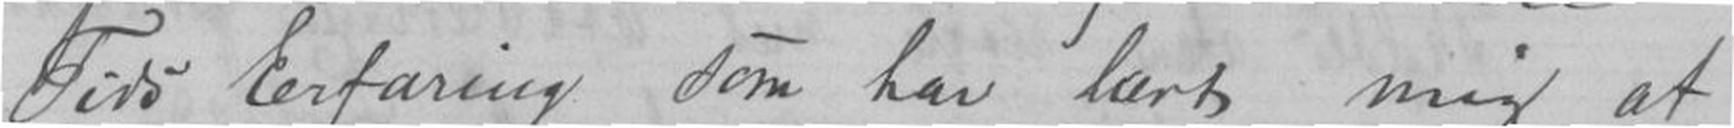Tids Erfaring som har lært mig at
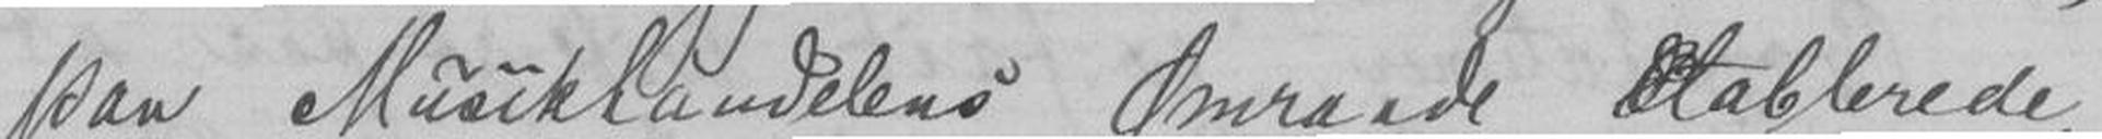paa Musiklandenes Omraade Etablerede
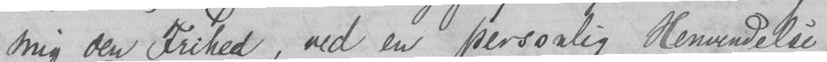mig den Frihed, ved en personlig Henvendelse
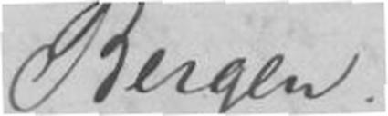Bergen.
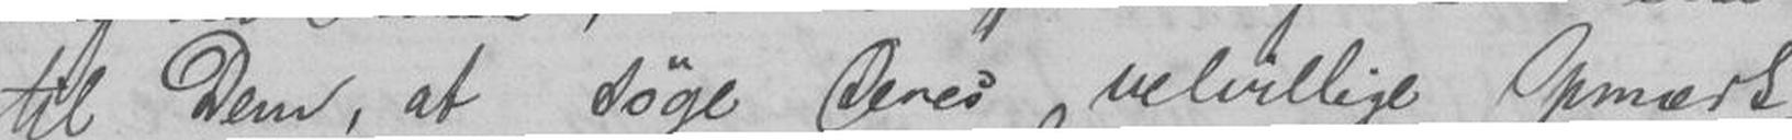til Dem, at søge Deres velvillige Opmærk-
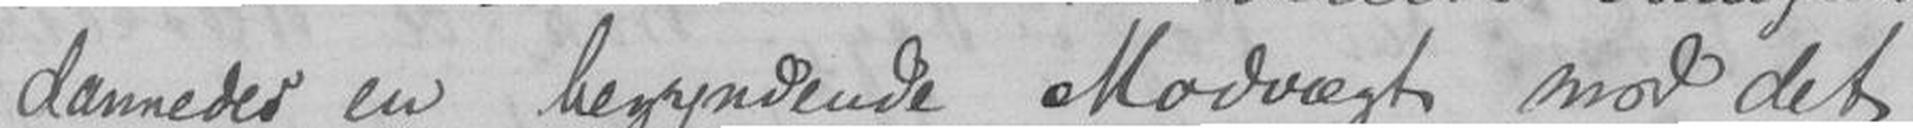dannedes en begyndende Modvægt mod dets
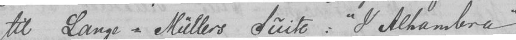til Lange - Müllers Suite: "S Alhambra".
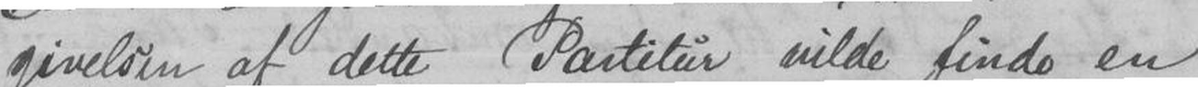givelsen af dette Partitur vilde finde en
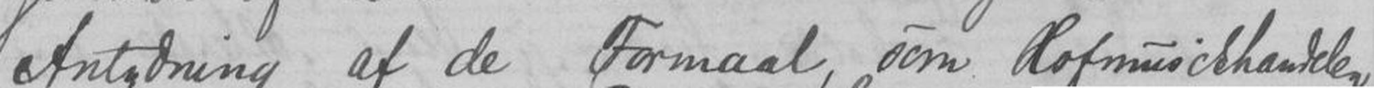Antydning af de Formaal, som Hofmusikhanten
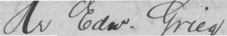Hr Edv. Grieg
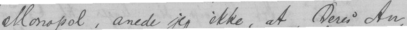Monopol, anede jeg ikke, at Deres An-
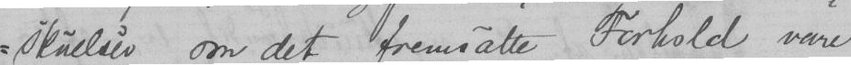skuelser om det fremsatte Forhold være
